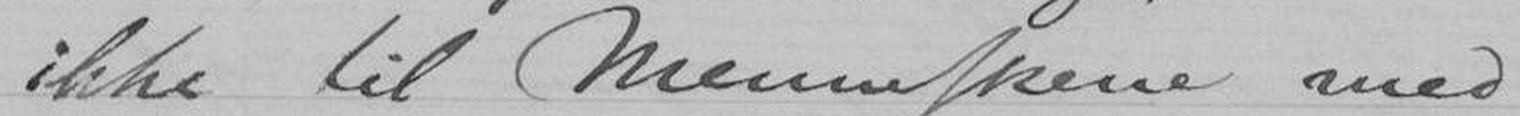ikke til Menneskene med,
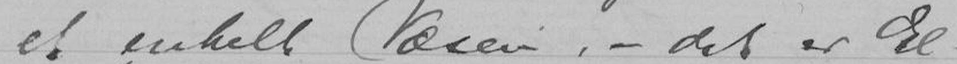et enkelt Væsen, - det er El-
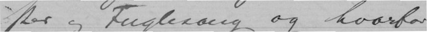ster og Fuglesang og hvorfor
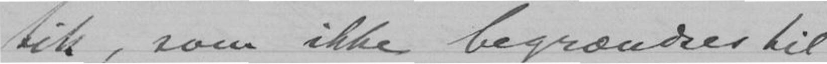tik, som ikke begrændses til
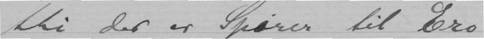thi der er Sporer til Ero-
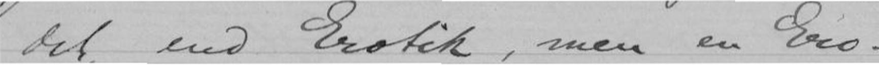det end Erotik, men en Ero-
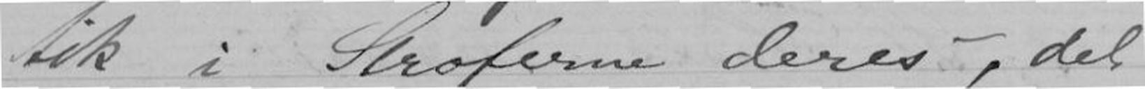tik i Stroferne deres, det
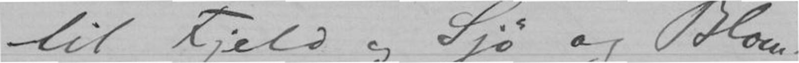til Fjeld og Sjø og Blom-
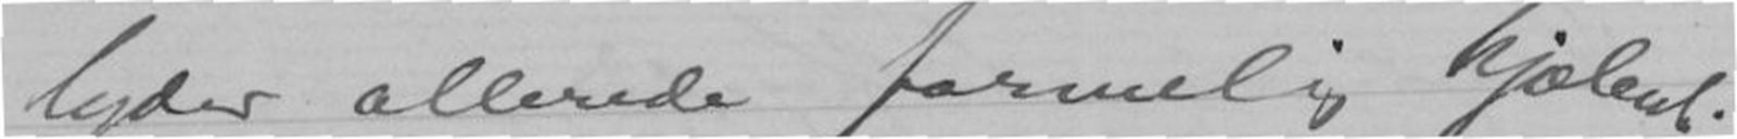lyder allerede formelig kjælent.
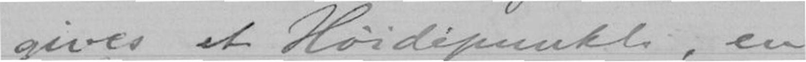gives et Høidepunkt, en
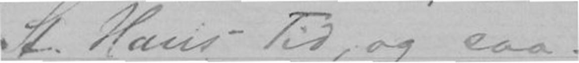St. Hans Tid, og saa
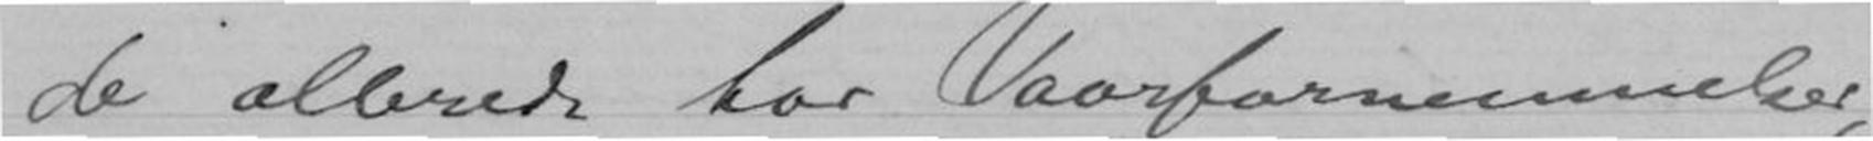de allerede har Vaarfornemmelser,
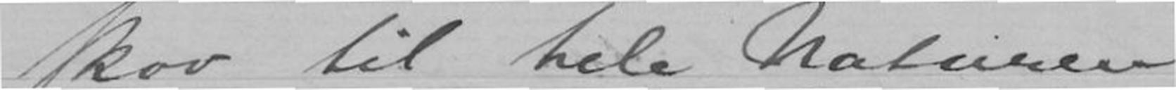skov til hele Naturen
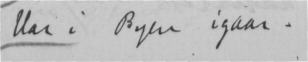Var i Byen igaar.
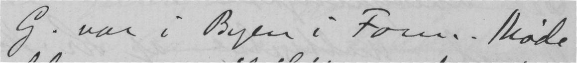G. var i Byen i Form.. Møde
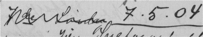7.5.04
